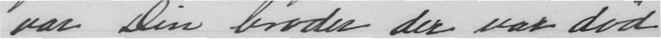var Din broder der var død,
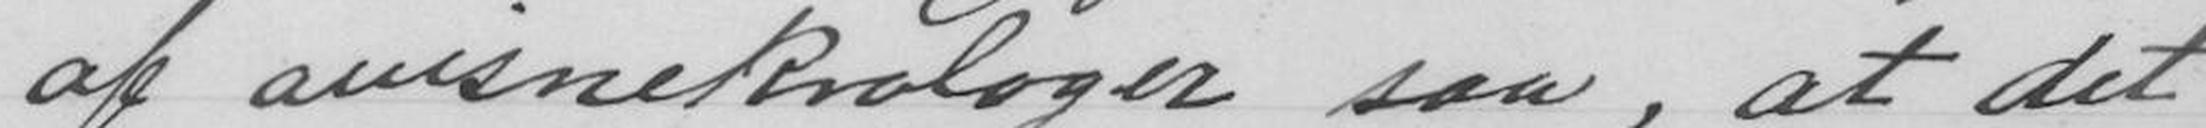af avisnekrologer saa, at det
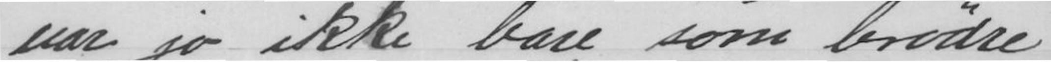var jo ikke bare som brødre
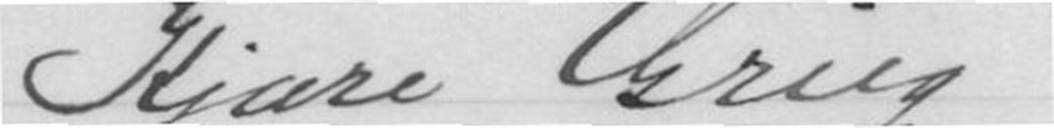Kjære Grieg!
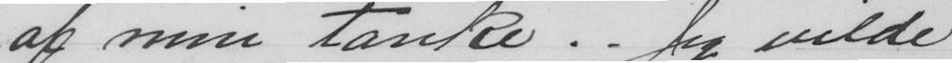af min tanke. - Jeg vilde,
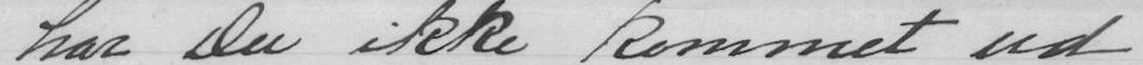har Du ikke kommet ud
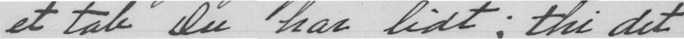et tab Du har lidt; thi det
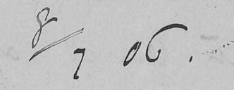8/7 06.
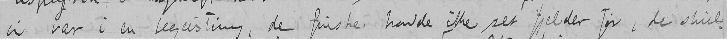vi var i en begeistring, de finske havde ikke set fjelder før, de skul-
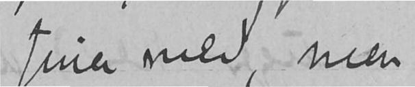fine med, men
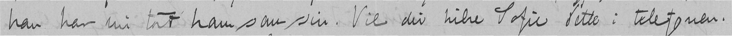han har nu tat ham som sin. Vil du hilse Sofie dette i telefonen.
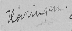Høvringen.
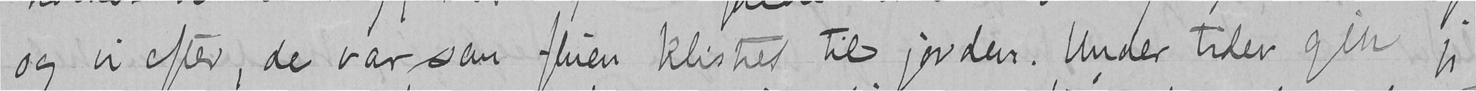og vi efter, de var som fluer klistret til jorden. Under tiden gik jeg
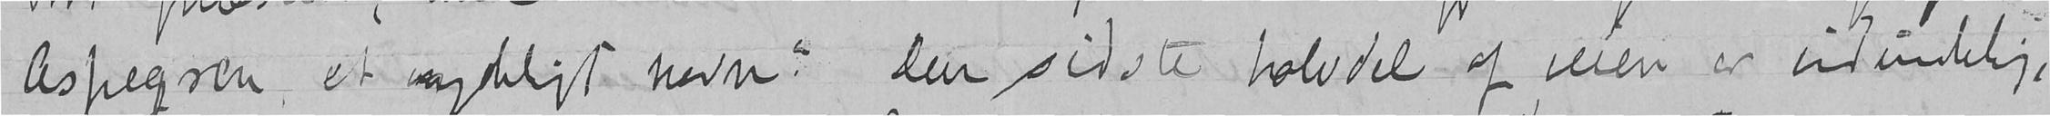Aspegren, et nydeligt navn. Den sidste halvdel af veien er vidunderlig,
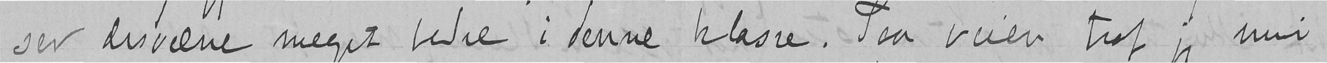ser desuden meget bedre i denne klasse. Paa veien traf jeg min
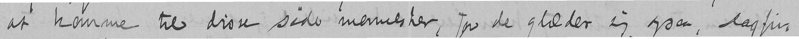at komme til disse søde mennesker, for de glæder sig ogsaa, Dagfin
